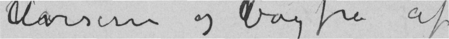Aviserne og bagfra af
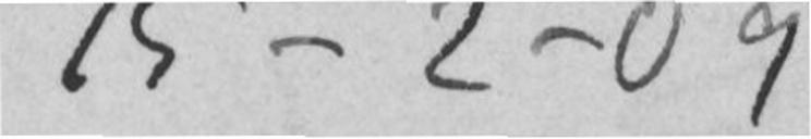15-2-09
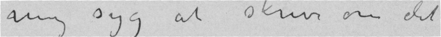mig syg at skrive om det
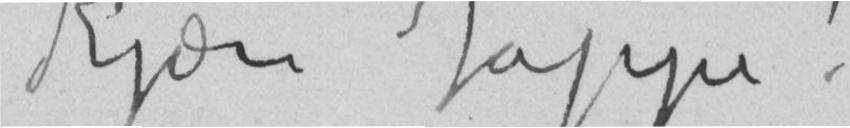Kjære Jappe!
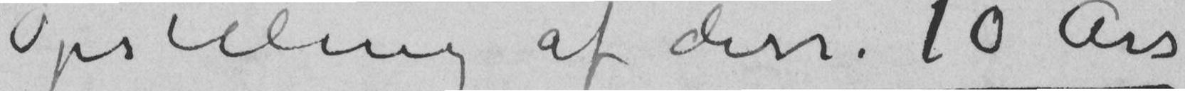Opstilling af disse 10 års
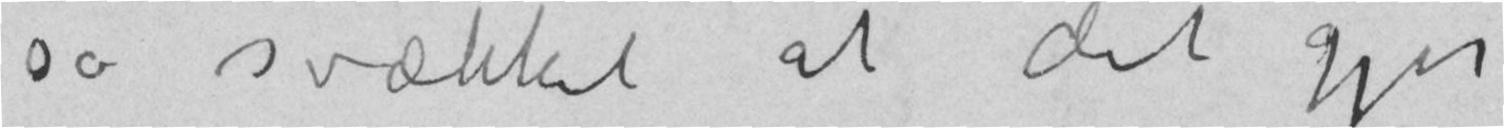så svækket at det gjør
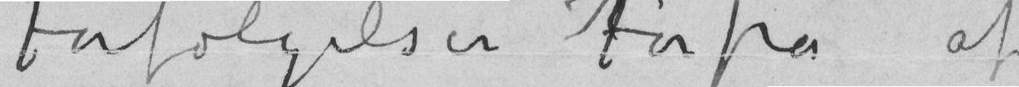Forfølgelser Forfra af
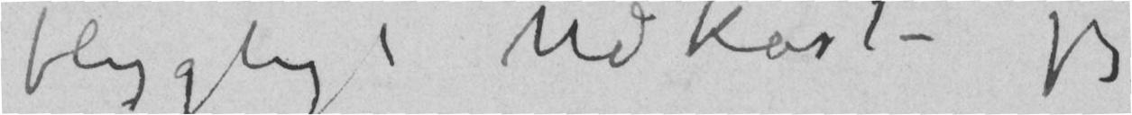flygtigt Udkast – jeg
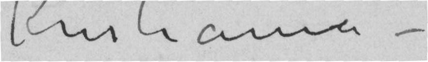Kristiania –
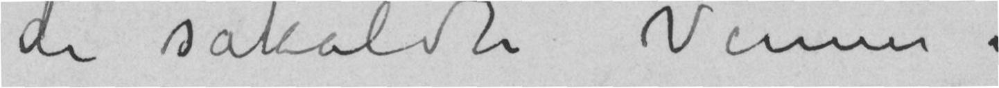de såkaldte Venner i
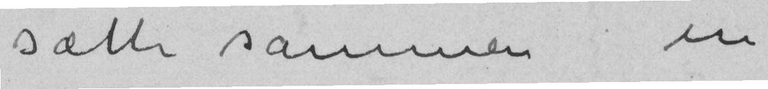sætte sammen en
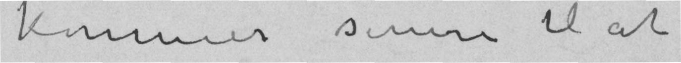kommer senere til at
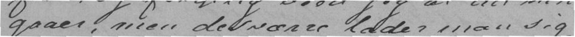gaaer, men desværre lader man sig
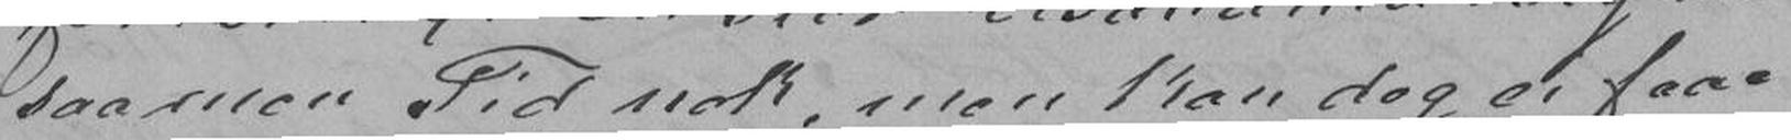saamen Tid nok, men kan dog ei faae
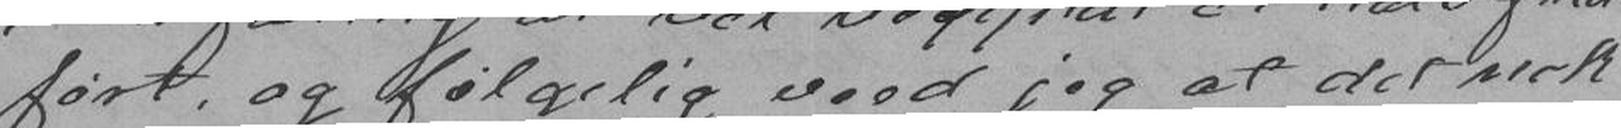ført, og følgelig veed jeg at det nok
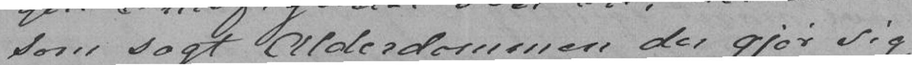som sagt Alderdommen der gjør sig
